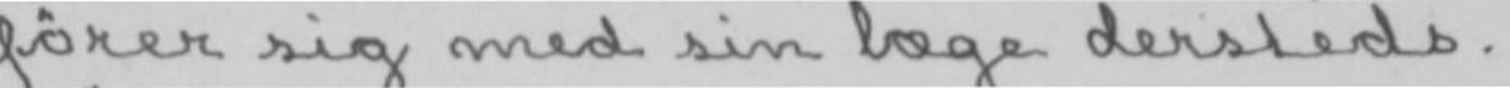fører sig med sin læge dersteds.
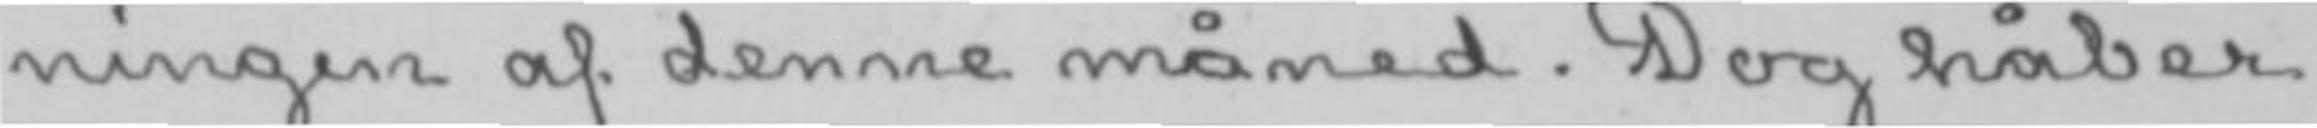ningen af denne måned. Dog håber
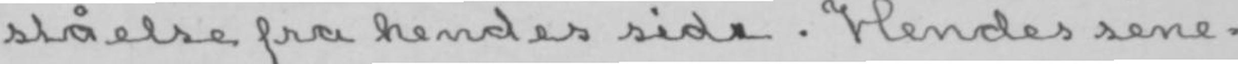ståelse fra hendes side. Hendes sene-
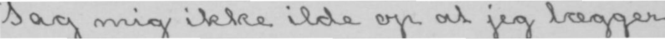Tag mig ikke ilde op at jeg lægger
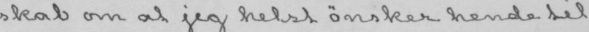skab om at jeg helst ønsker hende til
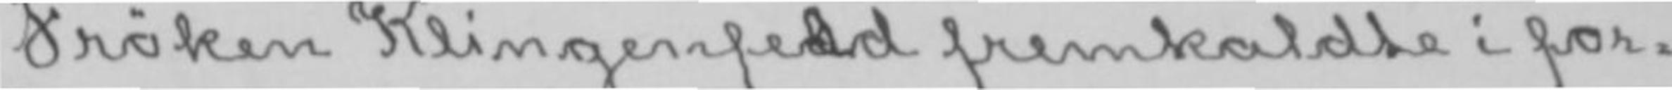Frøken Klingenfedld fremkaldte i for-
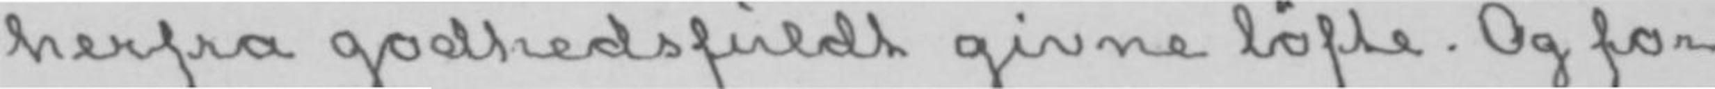herfra godhedsfuldt givne løfte. Og for
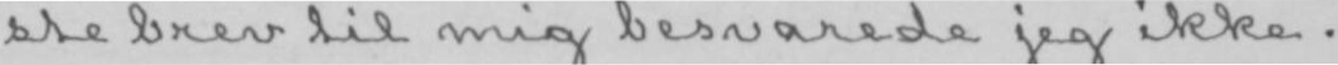ste brev til mig besvarede jeg ikke.
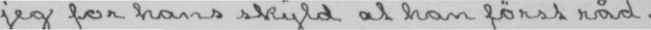jeg for hans skyld at han først råd-
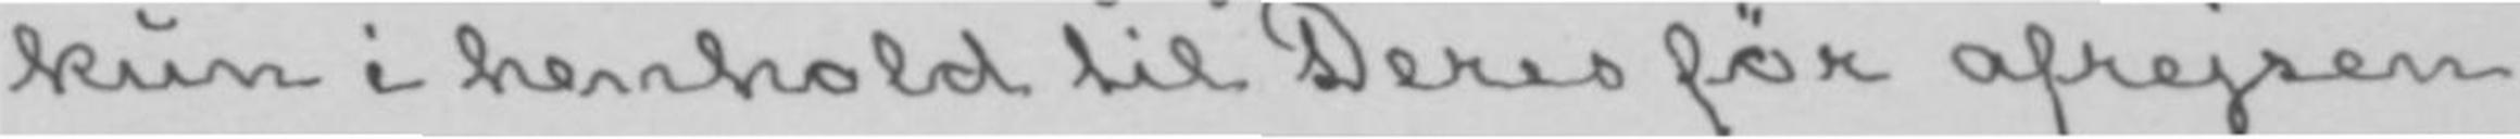kun i henhold til Deres før afrejsen
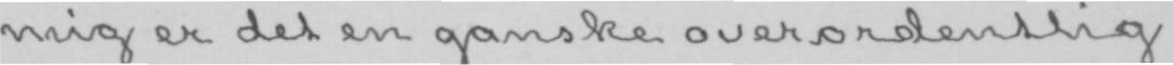mig er det en ganske overordentlig
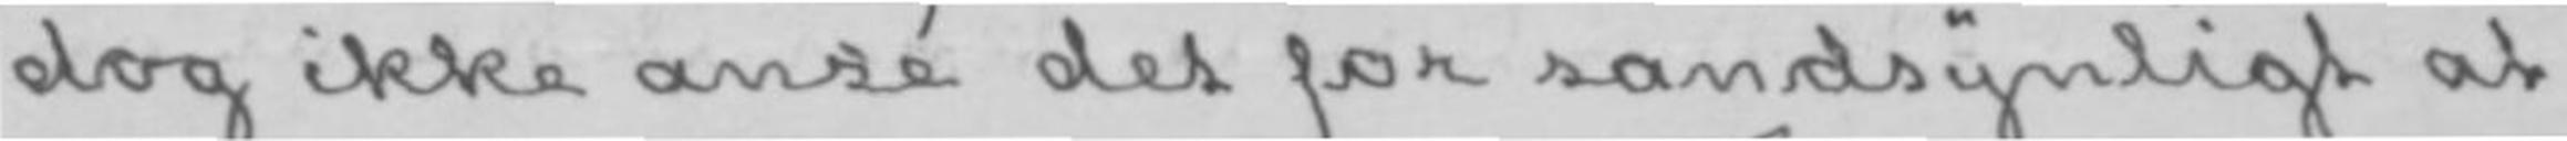dog ikke ansé det for sandsynligt at
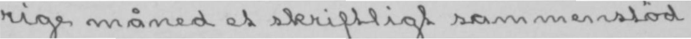rige måned et skriftligt sammenstød
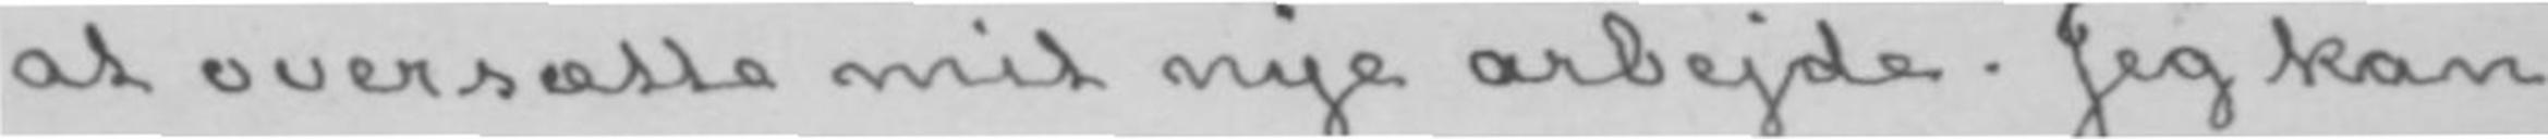at oversætte mit nye arbejde. Jeg kan
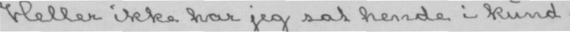Heller ikke har jeg sat hende i kund-
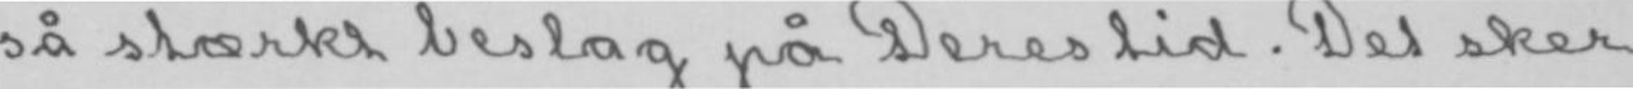så stærkt beslag på Deres tid. Det sker
